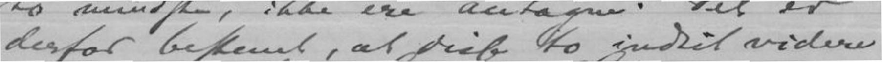derfor bestemt, at disse to indtil videre
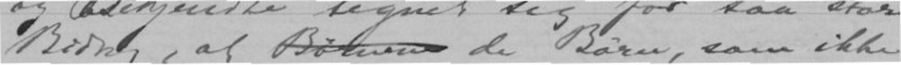Bidrag, at Børnene de Børn, som ikke
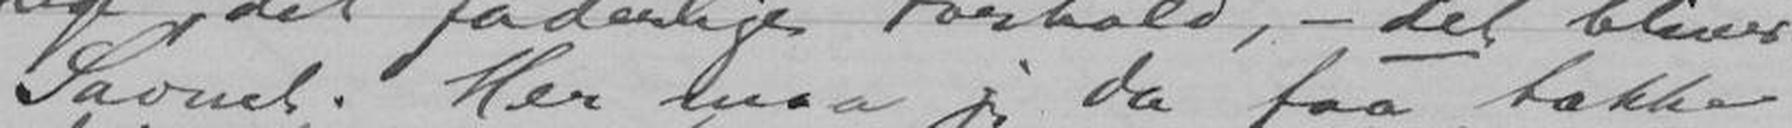Savnet. Her maa jeg da faa takke
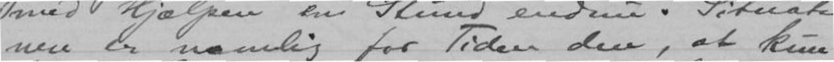nen er nemlig for Tiden den, at kun
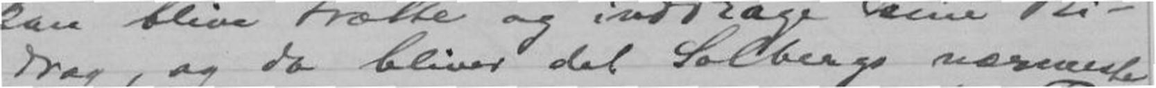drag, og da bliver det Solbergs nærmeste
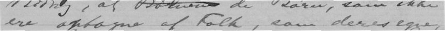ere optagne af Folk, som deres egne,
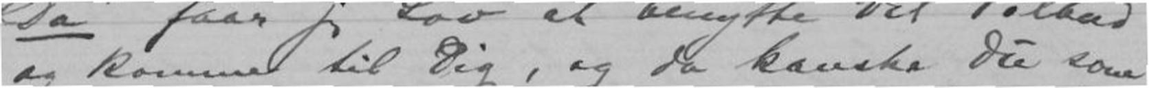og komme til Dig, og da kanske Du som
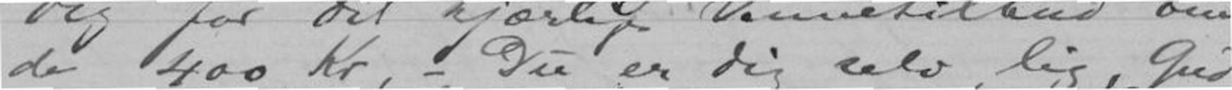de 400 Kr, - Du er Dig selv lig, Gud
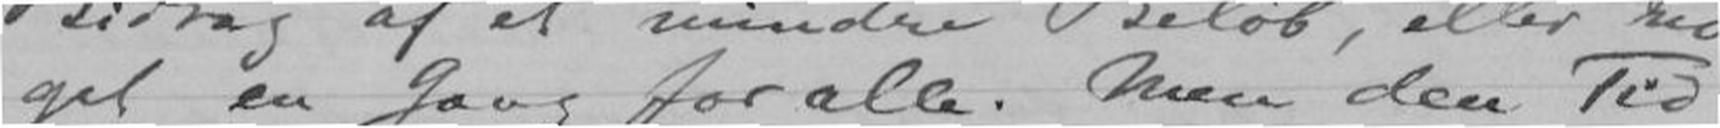get en Gang for alle. Men den Tid
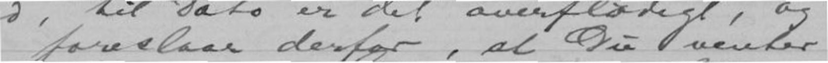jeg foreslaar derfor, at Du venter
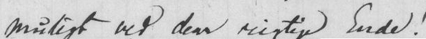muligt ved den rigtige Ende!
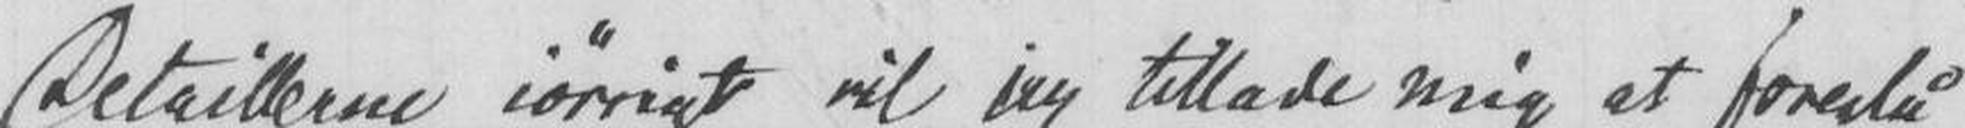Detaillerne iøørigt vil jeg tillade mig at foreslå
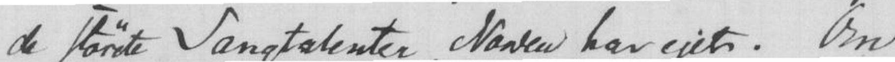de største Sangtalenter, Naaden har git. Om
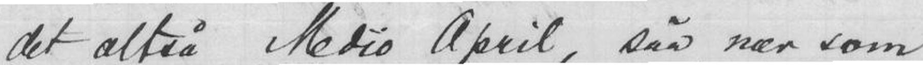det altså Medio April, saa nær som
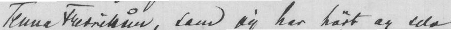Fenna Fredriksen, som jeg har hørt er selv
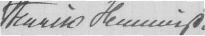Henrik Hennings
